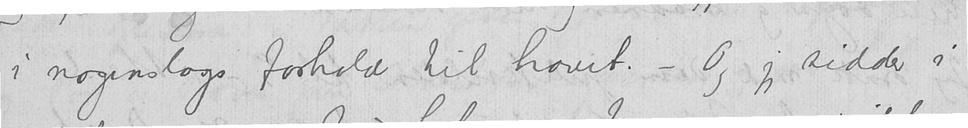i nogenslags forhold til havet. - Og jeg sidder i
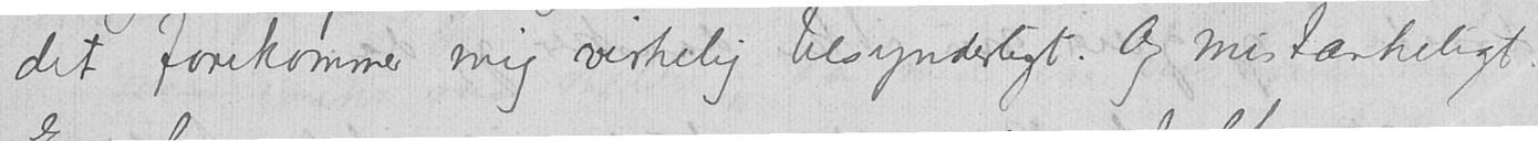det forekommer mig virkelig besynderligt. Og mistænkeligt.
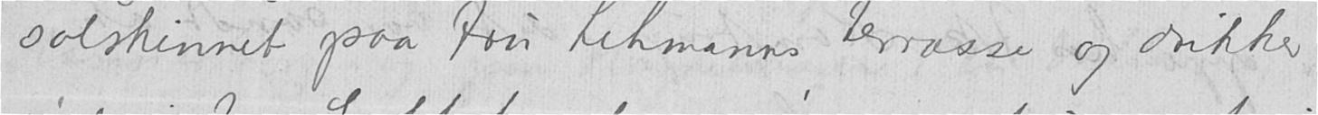solskinnet paa Fru Lehmanns terrasse og drikker
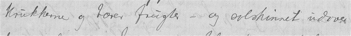krukkerne og bærer frugter - og solskinnet udover
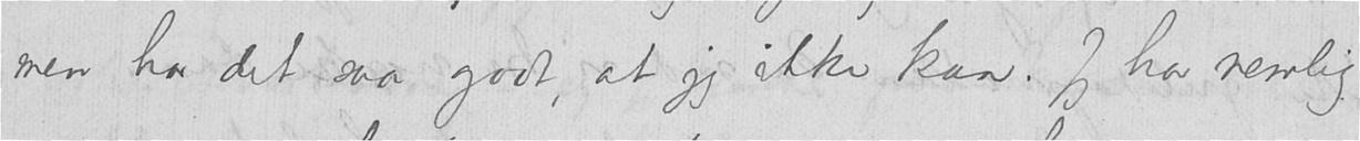men har det saa godt, at jeg ikke kan. Jeg har nemlig
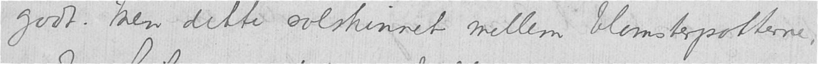godt. Men dette solskinnet mellem blomsterpotterne,
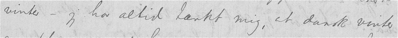vinter – jeg har altid tænkt mig, at dansk vinter
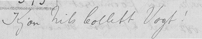Kjære Nils Collett Vogt!
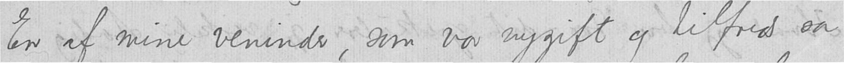En af mine veninder, som var nygift og tilfreds, sa
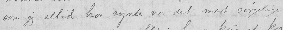som jeg altid har syntes var det mest sørgelige
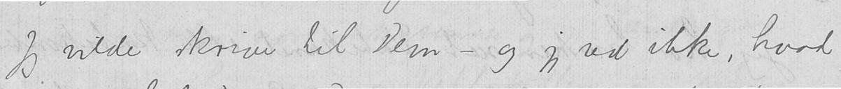Jeg vilde skrive til Dem - og jeg ved ikke, hvad
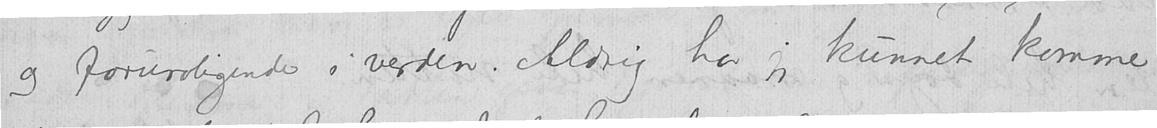og foruroligende i verden. Aldrig har jeg kunnet komme
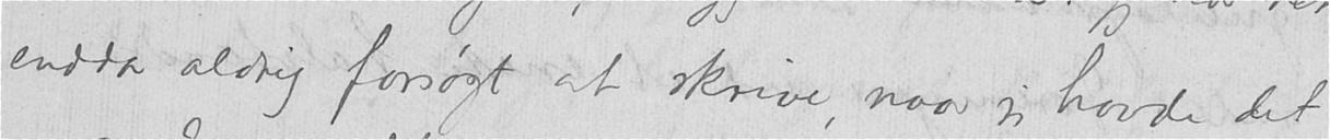endda aldrig forsøgt at skrive, naar jeg havde det
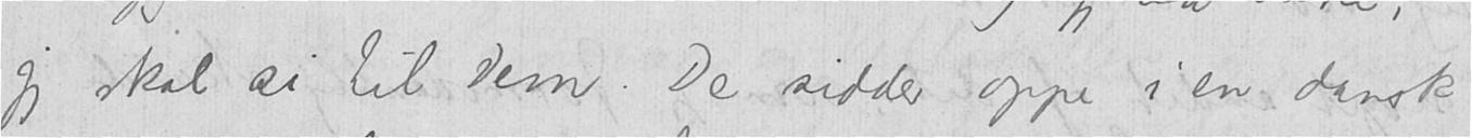jeg skal si til Dem. De sidder oppe i en dansk
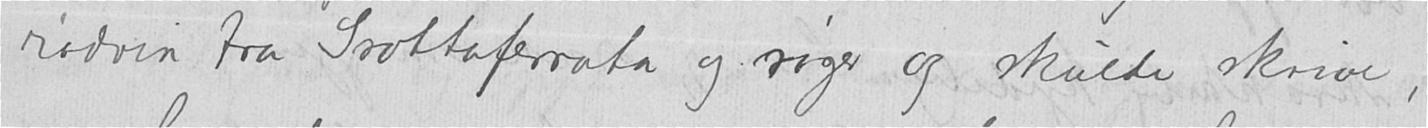rødvin fra Grottaferrata og søger og skulde skrive,
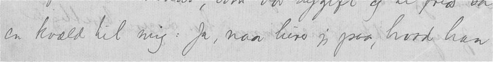en kvæld til mig. Ja, naar lurer jeg paa, hvad han
